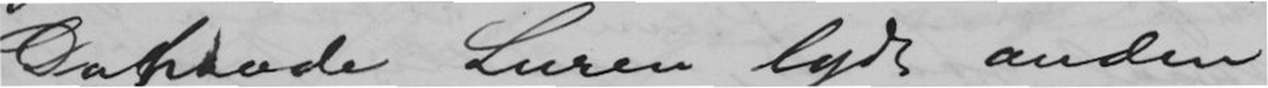Da havde Luren lydt anden
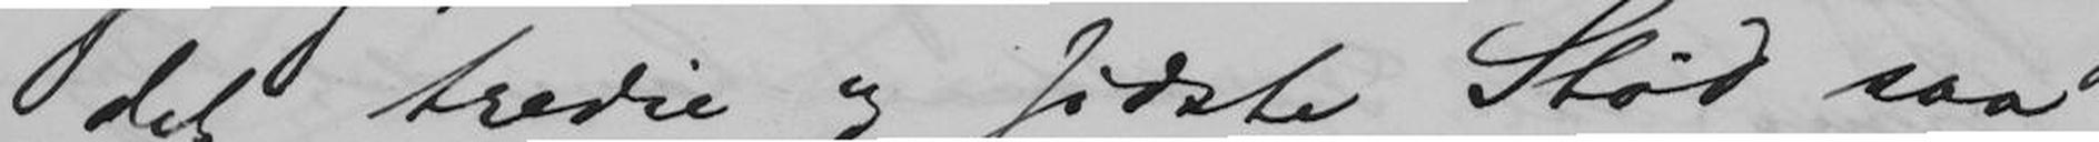det tredie og sidste Stød saa
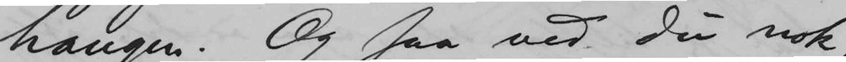haugen. Og saa ved du nok,
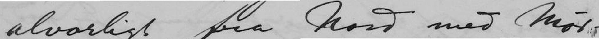alvorligt fra Nord med Mør-
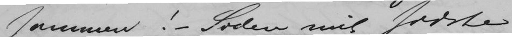sammen! - Siden mit sidste
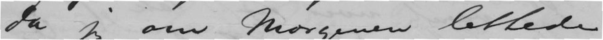da jeg om Morgenen lettede
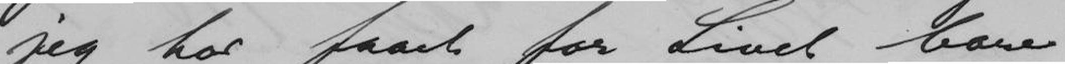jeg har faaet for Livet bare
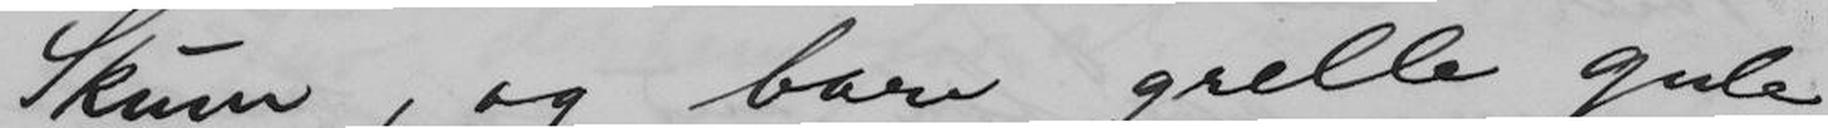Skum, og bare grelle gule
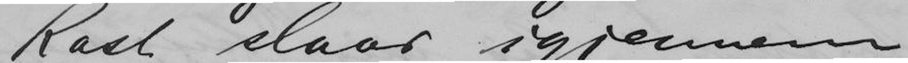Kast slaar igjennem
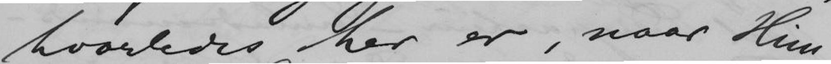hvorledes her er, naar Him-
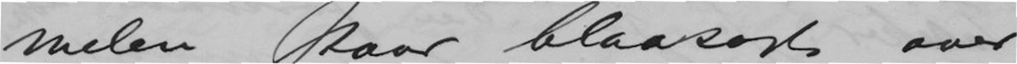melen staar blaasort over
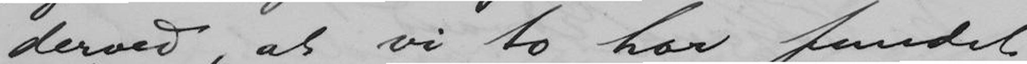derved, at vi to har fundet
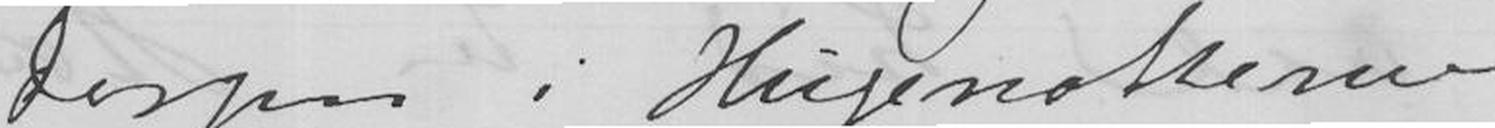derpaa i Hugenotterne
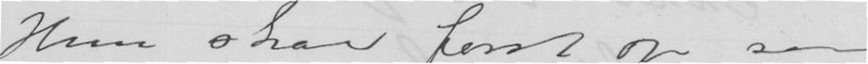Hun skal først op som
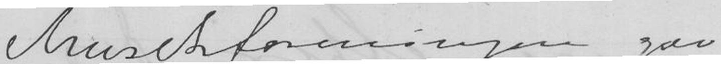Musikforeningen gav
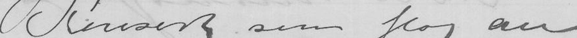Konsert som slog an
